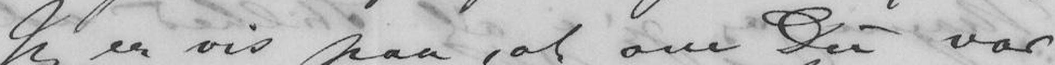Jeg er vis paa, at om Du var
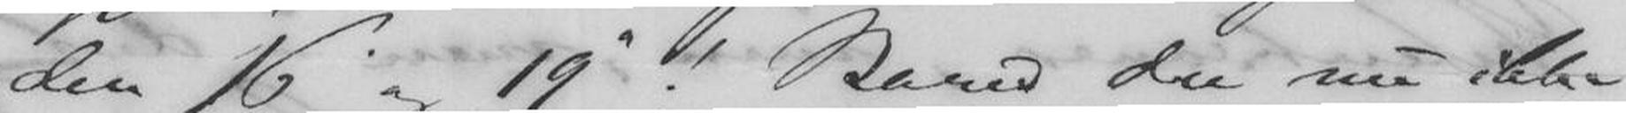den 16 og 19de! Bare du nu ikke
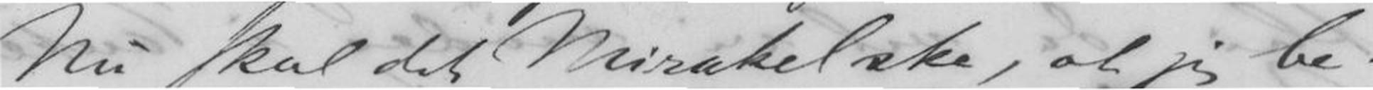Nu skal det Mirakel ske, at jeg be-
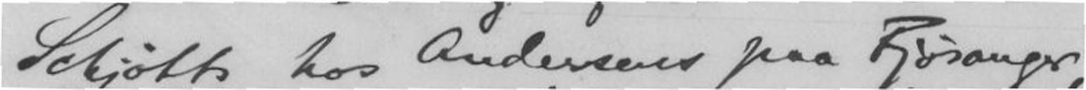Schjøtts hos Andersens paa Fjøsanger,
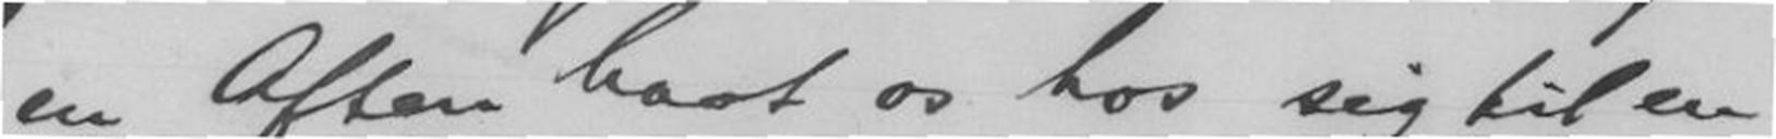en Aften havt os hos sig til en
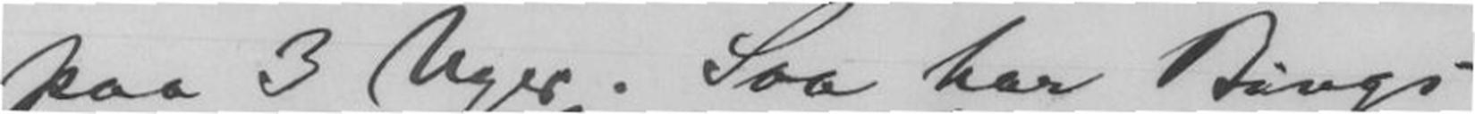paa 3 Uger. Saa har Bings
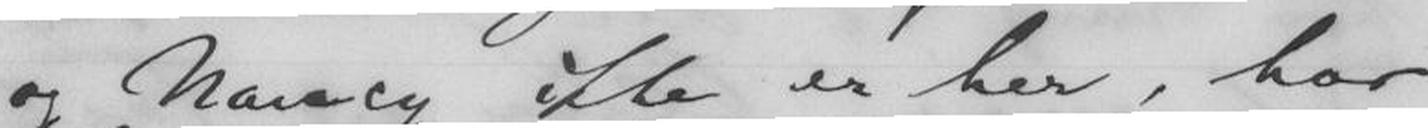og Nancy ikke er her, har
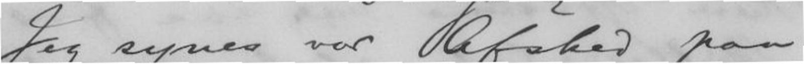Jeg synes vor Afsked paa
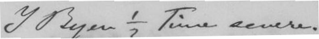I Byen ½ Time senere.
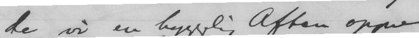de vi en hyggelig Aften oppe
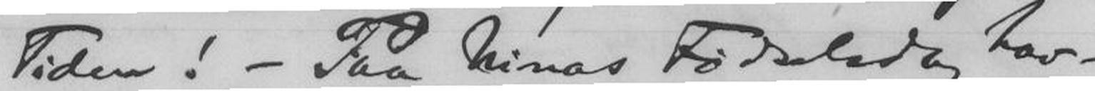Tiden! - Paa Ninas Fødselsdag hav-
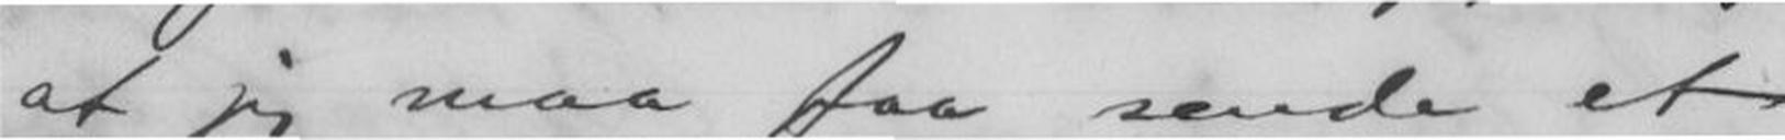at jeg maa faa sende et
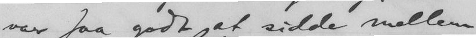var saa godt at sidde mellem
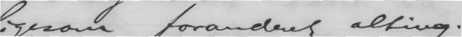ligesom forandret alting.
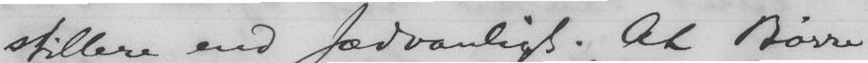stillere end sædvanligt. At Børre
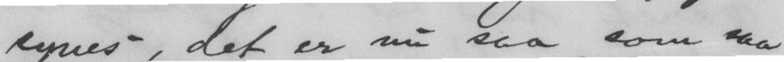synes, det er nu saa som saa
